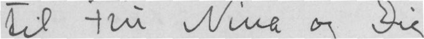til Fru Nina og Dig
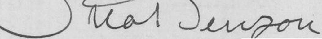Otto Benzon
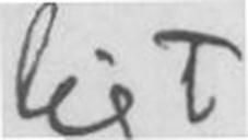ligt.
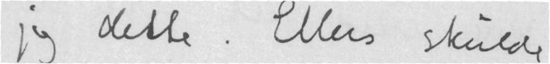jeg dette. Ellers skulde
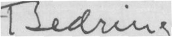Bedring!
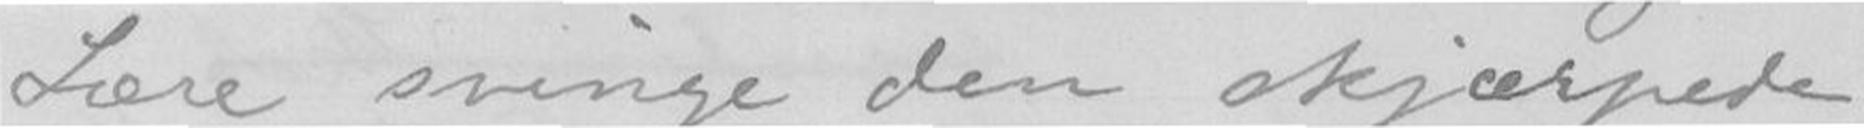Lære svinge den skjærpede
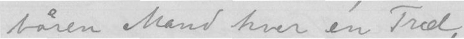båren Mand hver en Træl,
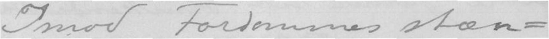Imod Fordommes Stæn-
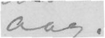Aag.
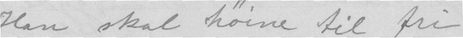Han skal høine til fri
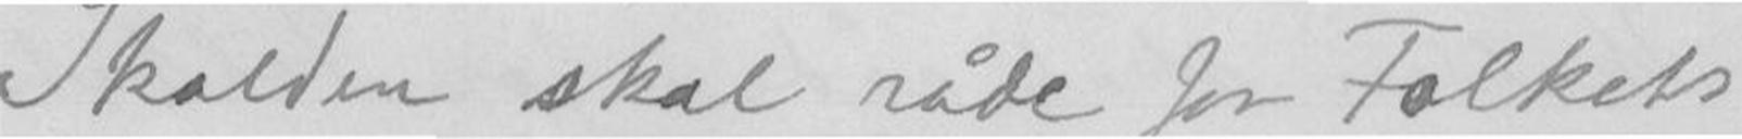Skalden skal råde for Folkets
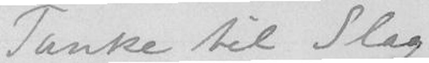Tanke til Slag
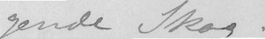gende Skog.
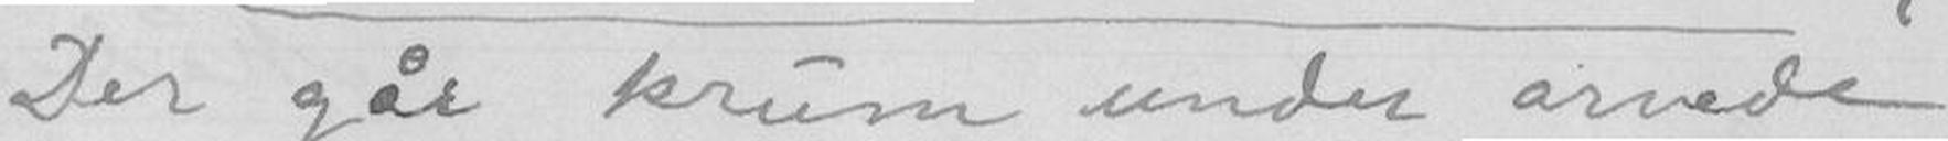Der går krum under arvede
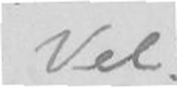Vel!
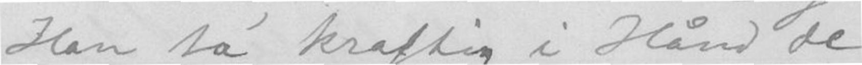Han ta' kraftig i Hånd de
